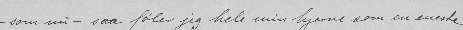- som nu - saa føler jeg hele min hjerne som en eneste
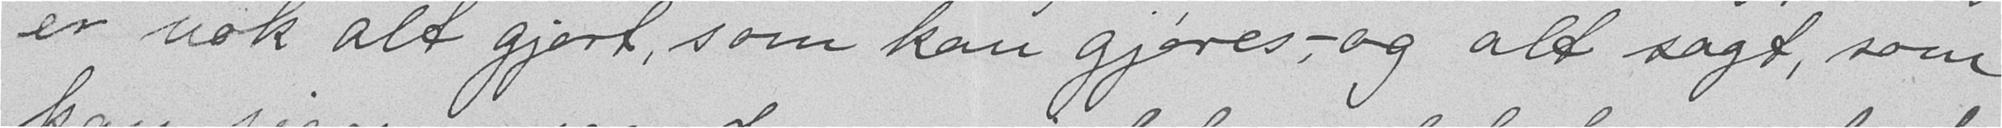er nok alt gjort, som kan gjøres, - og alt sagt, som
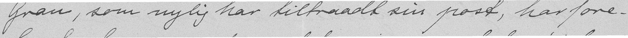Gran, som nylig har tiltraadt sin post, har fore-
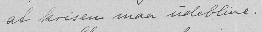at krisen maa udeblive.
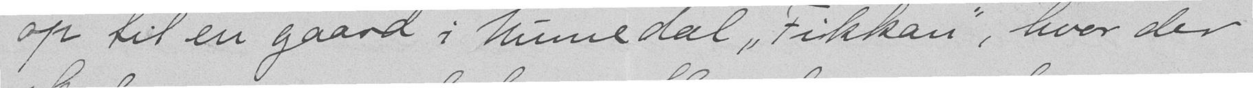op til en gaard i Numedal "Fikkan", hvor der
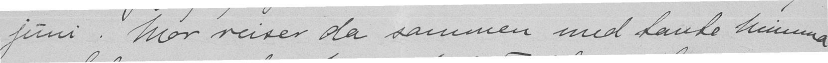juni. Mor reiser da sammen med tante Mimma
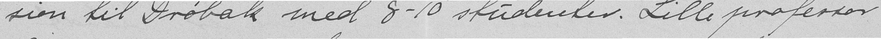sion til Drøbak med 8-10 studenter. Lille professor
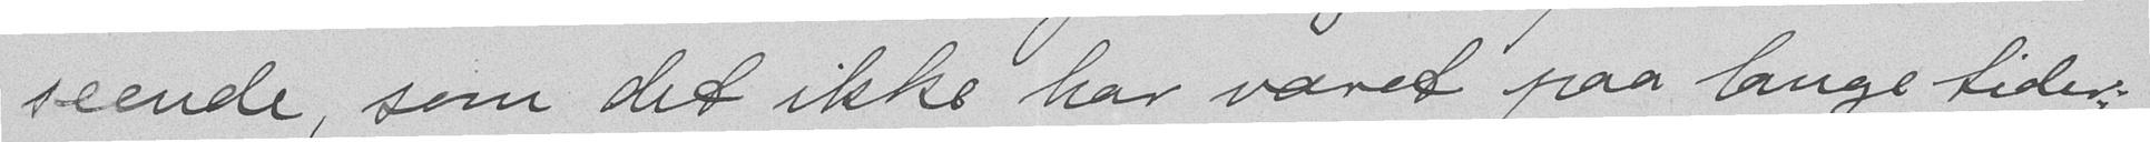seende, som det ikke har været paa lange tider;
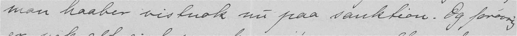man haaber vistnok nu paa sanktion. Og forøvrig
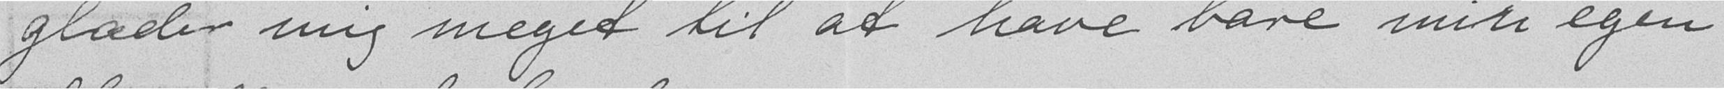glæder mig meget til at have bare min egen
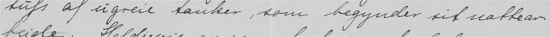tufs af ugreie tanker, som begynder sit nattear-
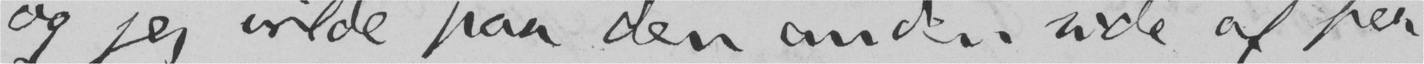og jeg vilde paa den anden side af per-
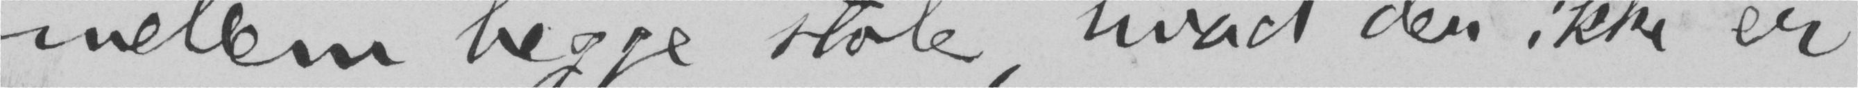mellem begge stole, hvad der ikke er
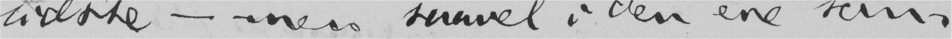sidste – men saavel i den ene som
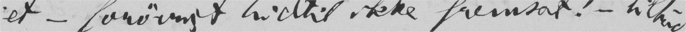et – forøvrigt hidtil ikke fremsat! – tilbud
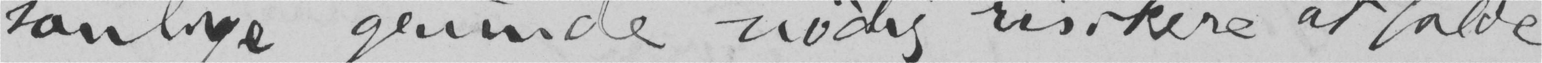sonlige grunde nødig risikere at falde
